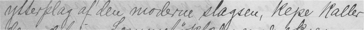ytterplag af den moderne slagsen, kepe kaller
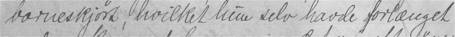barneskjørt, hvilket hun selv havde forlænget
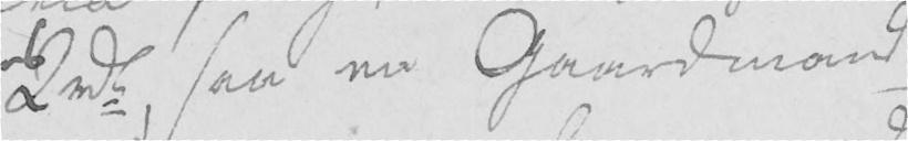2 rd saa en Gaardmand
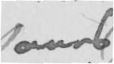omtr
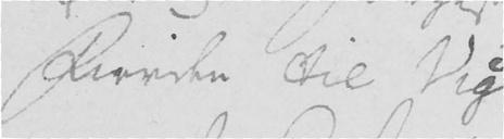Fiorden til Vig
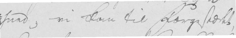sund; vi kom til Færgestædet,
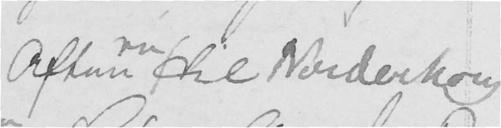Aften til Norderhoug
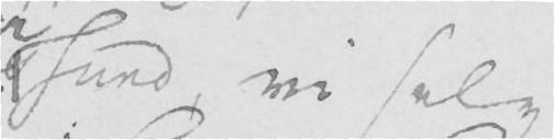sund, vi selv
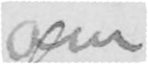om
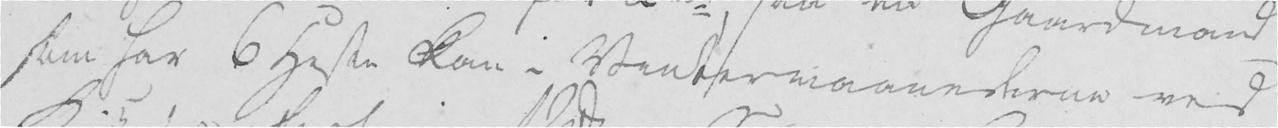som har 6 Heste kan i Vintermaanederne ved
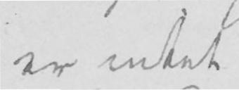er intet
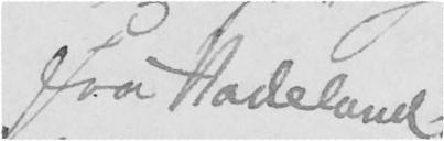fra Hadeland
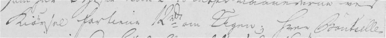Kiørsel fortiene 12 rde om Ugen; hver Bouteille
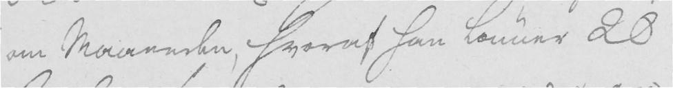om Maaneden, hvoraf han lønner 20
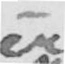er
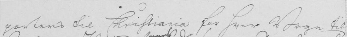porteres til Christiania for hver Vogn til
